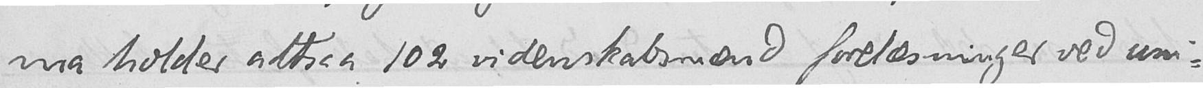ma holder altsaa 102 videnskabsmænd forelæsninger ved uni-
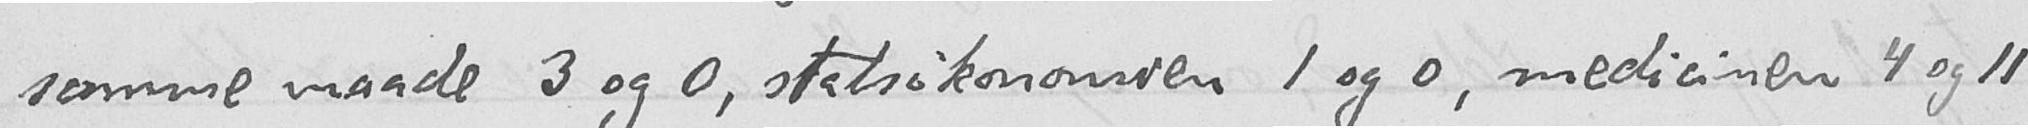samme maade 3 og 0, statsøkonomien 1 og 0, medicinen 4 og 11
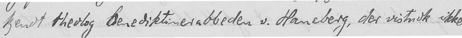kjendt theolog benediktinerabbeden v. Haneberg, der vistnok ikke
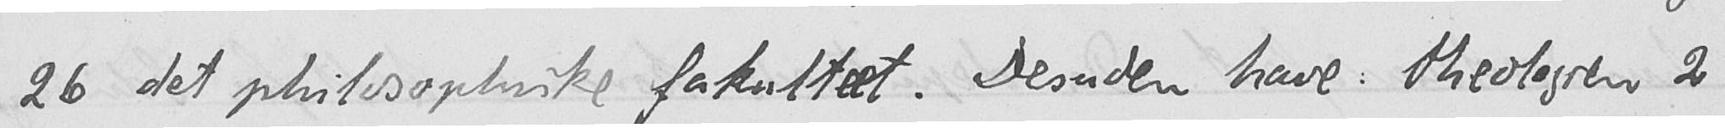26 det philosophiske fakultet. Desuden have theologien 2
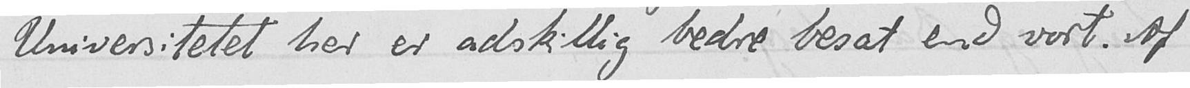Universitetet her er adskillig bedre besat end vort. Af
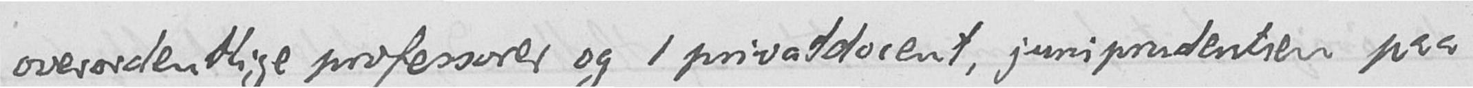overordentlige professorer og 1 privatdocent, jurisprudentsen paa
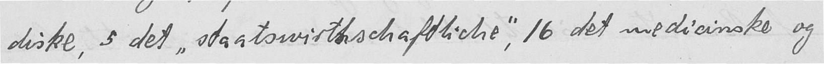diske, 5 det "staatswisthschaftliche" 16 det medicinske og
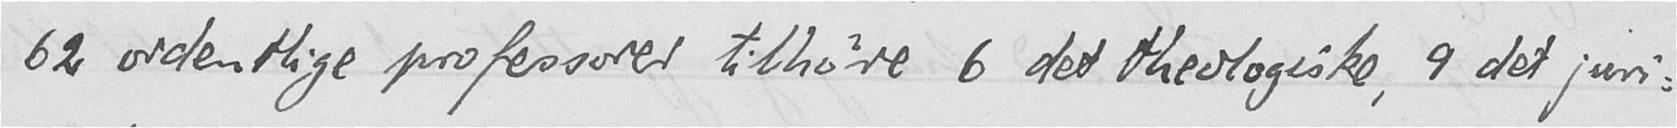62 ordentlige professorer tilhøre 6 det theologiske, 9 det juri-
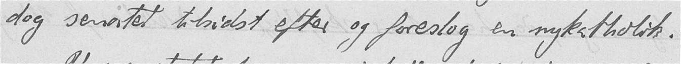dog senatet tilsidst efter og foreslog en nykatholik.
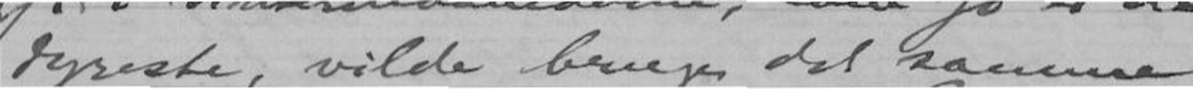dyreste, vilde bruge det samme
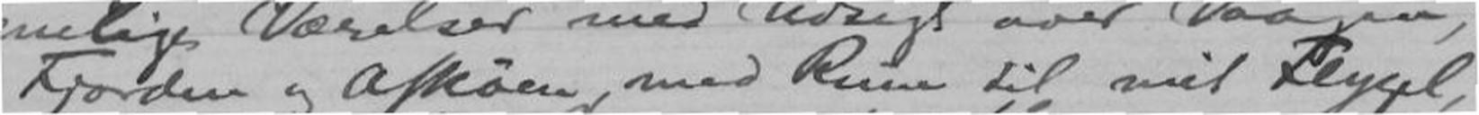Fjorden og Askøen, med Rum til mit Flygel,
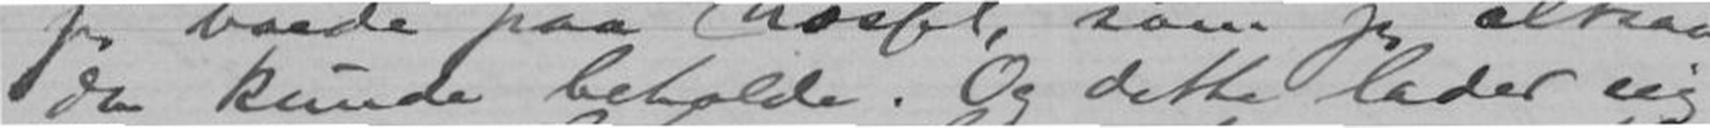da kunde beholde. Og dette lader sig
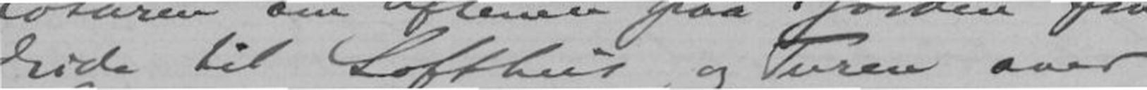Eid til Lofthus, og Turen over
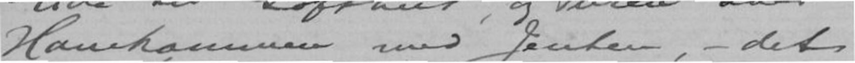Hanekammen med Jenten, - det
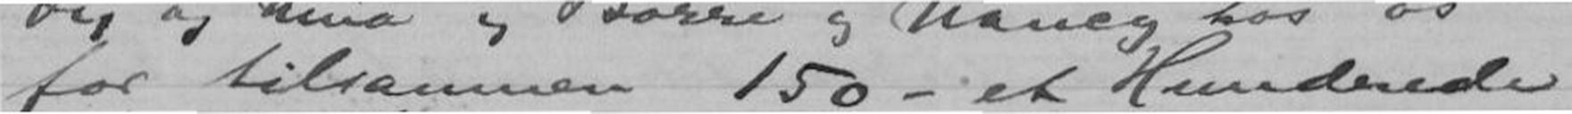for tilsammen 150 - et Hundrede
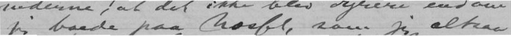jeg boede paa Næsset, som jeg altsaa
